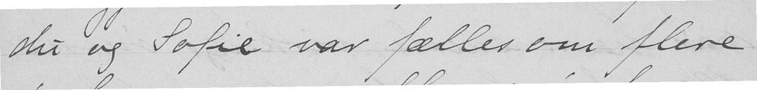du og Sofie var fælles om flere
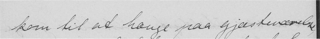kom til at hænge paa gjæsteværelse,
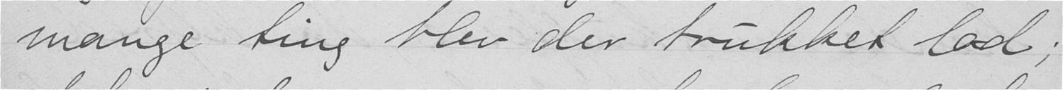mange ting blev der trukket lod;
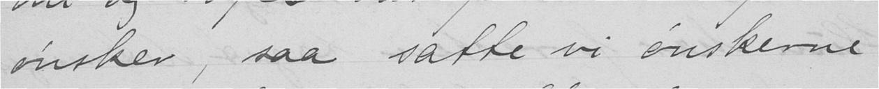ønsker, saa satte vi ønskerne
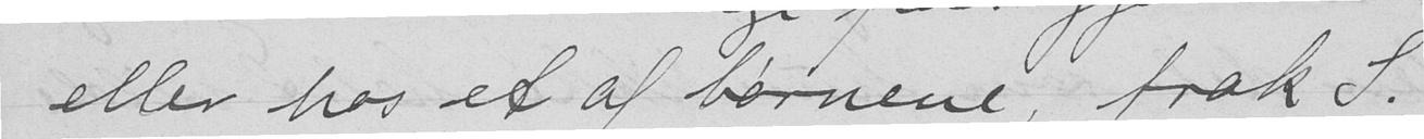eller hos et af børnene, trak S.
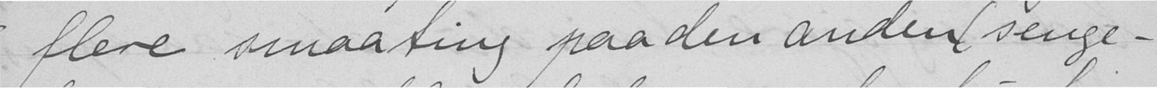flere smaating paa den anden (senge-
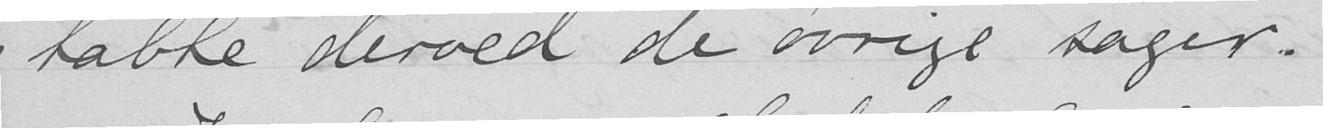tabte derved de øvrige sager.
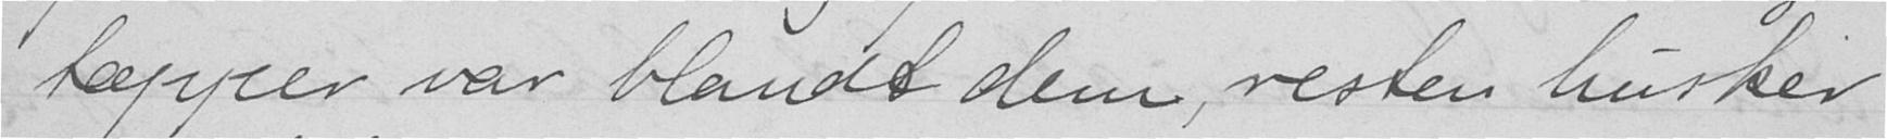tæpper var blandt dem, resten husker
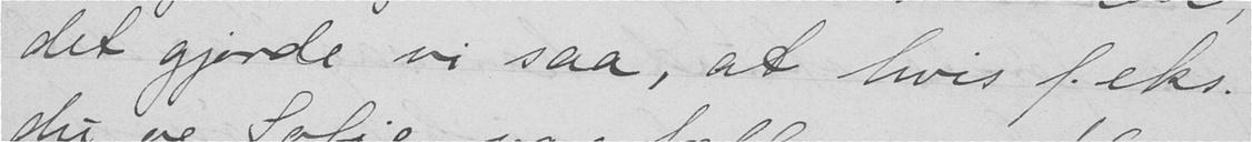det gjorde vi saa, at hvis f.eks.
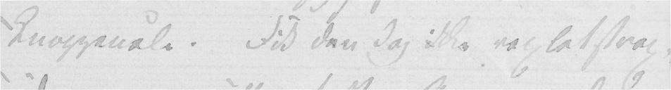Knappenåle. Fik den dog ikke vexlet strax
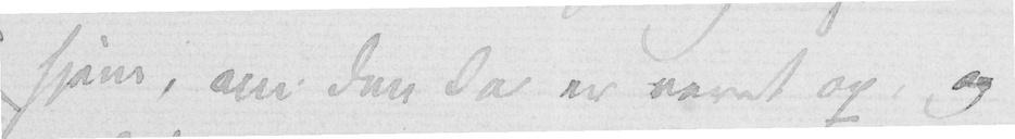hjem, om den da er varet op, og
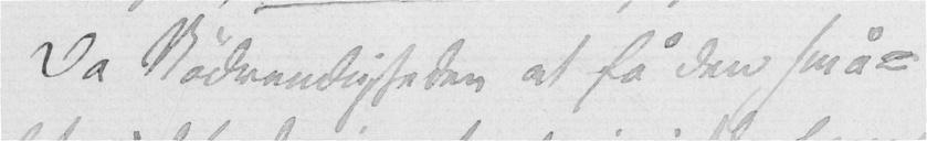Da Nødvendigheden at få den små-
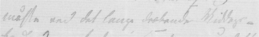måske ved det lange dræbende Middags-
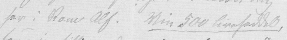her i Rom Alf. Min 500 Livsseddel,
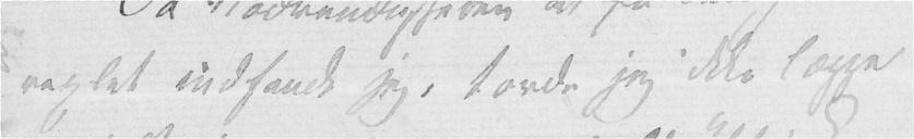vexlet indfandt sig, torde jeg ikke lægge
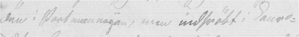den i Portmonnayen, men indsvøbt i Konvo-
